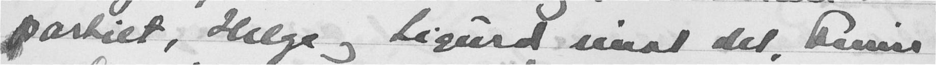partiet, Helge og Sigurd imot det, Finne
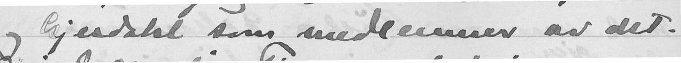og Gjesdahl som medlemmer av det.
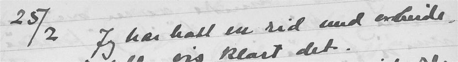25/2 Jeg har hatt en rid med arbeide
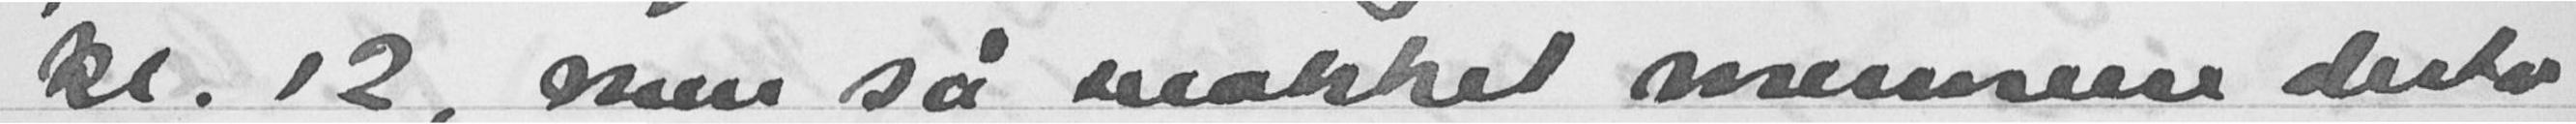kl. 12, men så snakket mennene desto
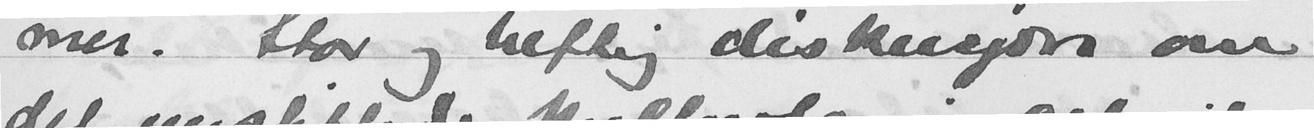mer. Stor og heftig diskusjon om
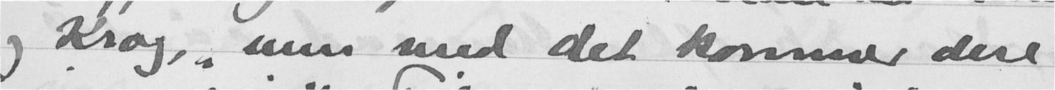og Krog, men med det kommer dere
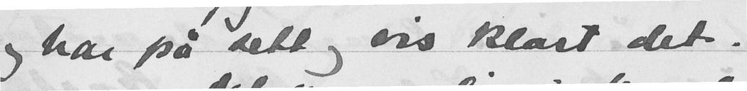og har på sett og vis klart det.
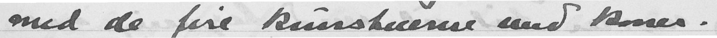med de fire kunstnerne med koner.
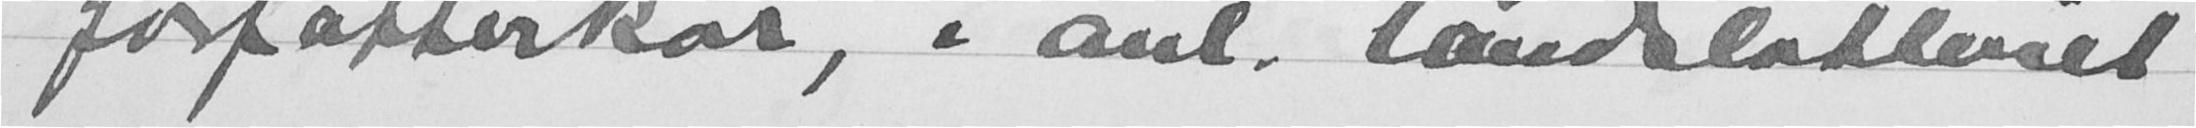forfatterkår, i anl. landslotteriet
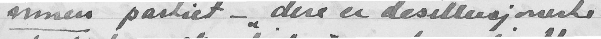innen partiet - "dere er desillusjonerte
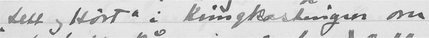"Sett og Hørt" i Kringkastingen om
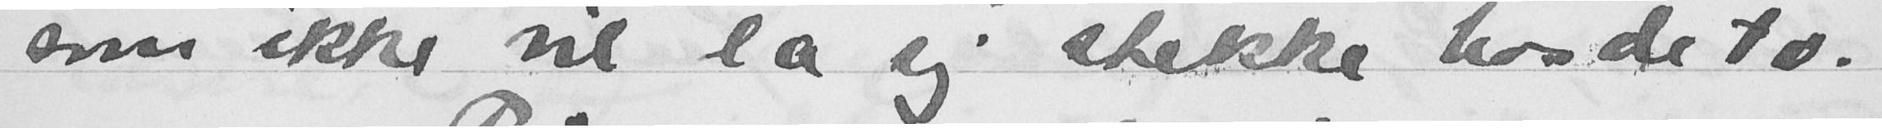som ikke vil la sig slokke hos de to.
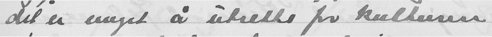det er meget å utsette for kulturen
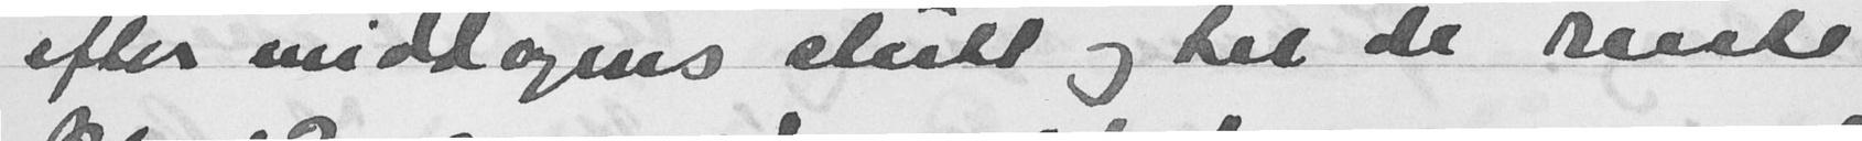efter middagens slutt og til de reiste
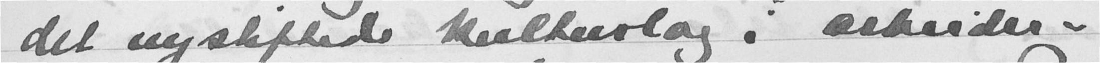det nystiftede kulturlag, arbeider-
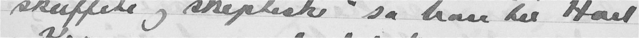skuffete og skeptiske", sa han til Hoel
